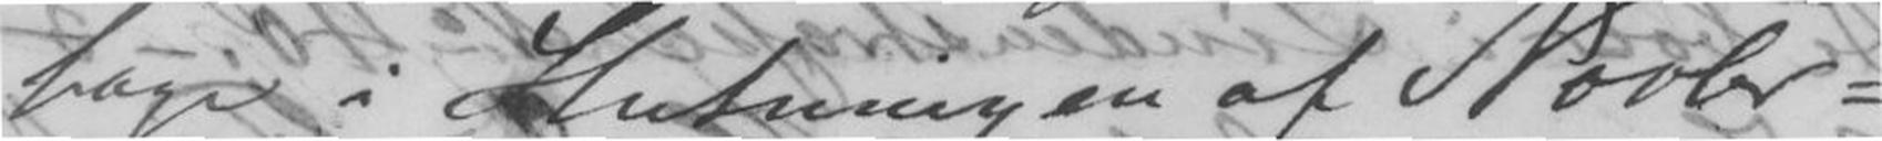bage i Slutningen af Novbr-
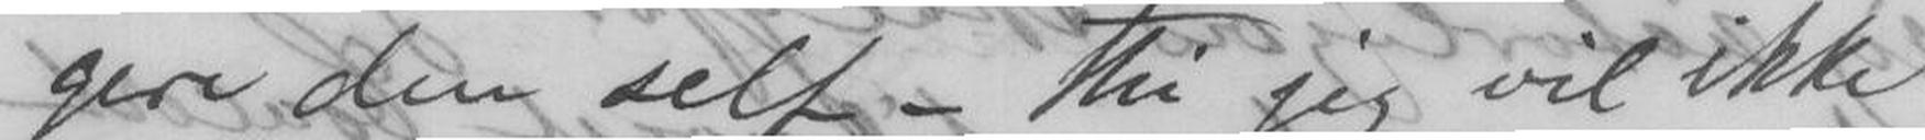gere den self - thi jeg vil ikke
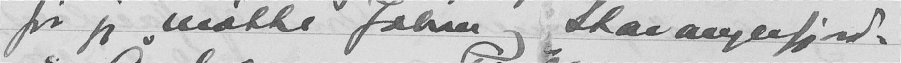før jeg møtte Johan og Stavangerfjord.
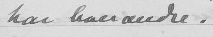har hverandre!
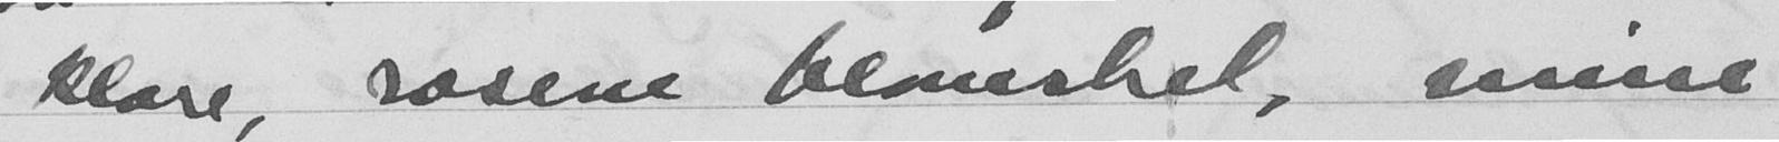klare, rosene blomstret, mine
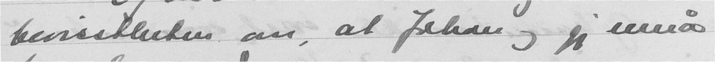bevisstheten om, at Johan og jeg ennå
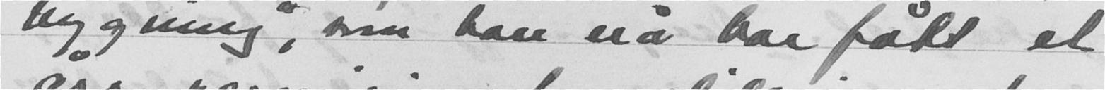bygning", som han nå har fått et
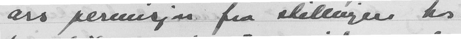års permisjon fra stillingen hos
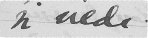jeg vilde.
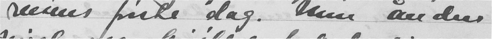reisens første dag. Men ånden
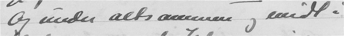Og under altsammen og midt i
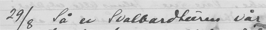29/8 Så er Svalbardturen vår
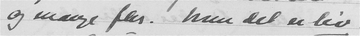og mange fler. Men det er liv.
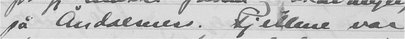på Åndalsness. Fjellene var
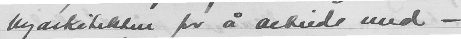byarkitekten for å arbeide med -
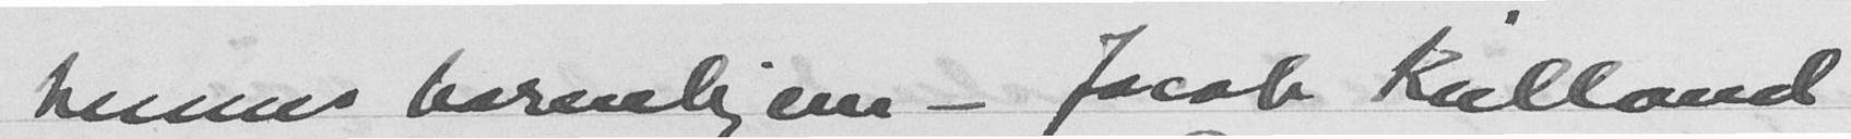hennes barnehjem - Jacob Kielland
